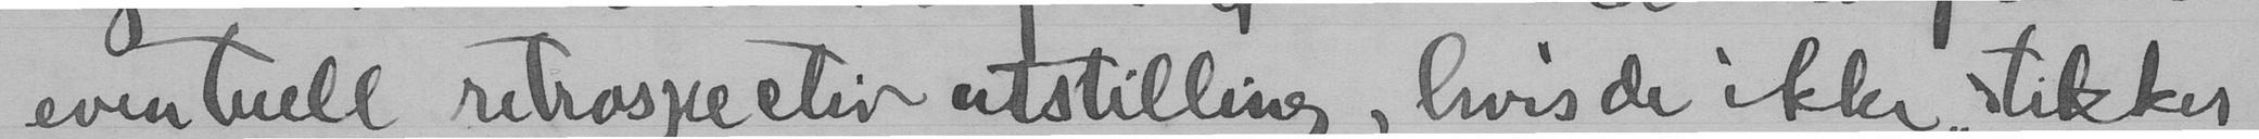eventuell retrospectiv utstilling, hvisde ikke "stikker"
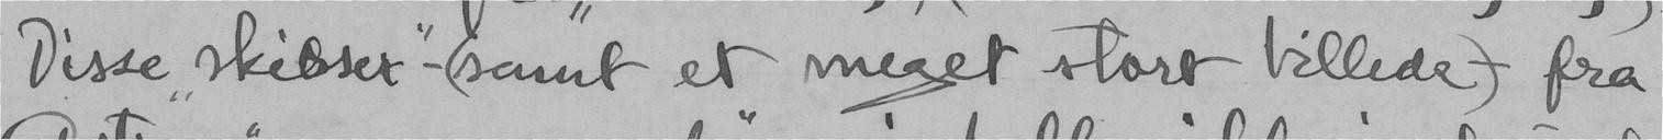Disse "skisser, - (samt et meget stort billede) fra
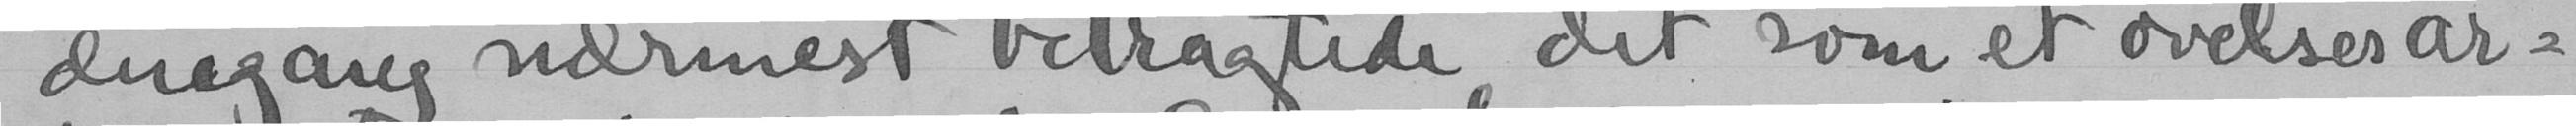dengang nærmest betragtede det som et øvelses ar-
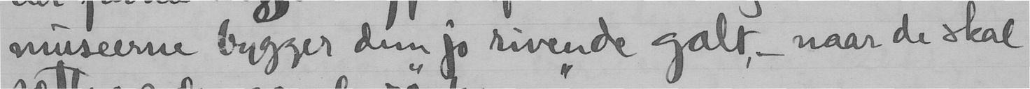museerne bygger dem jo rivende galt, - naar de skal
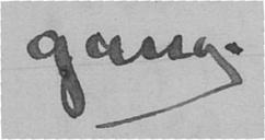gang.
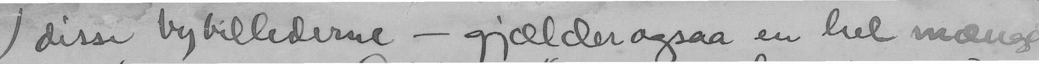disse bybillederne - gjælder ogsaa en hel mængde
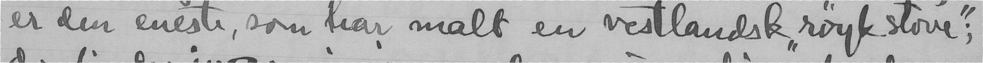er den eneste, som har malt en vestlandsk "røykstove";
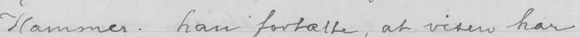Hammer; han fortalte, at visen har
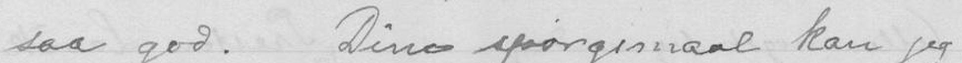saa god. Dine spørgsmaal kan jeg
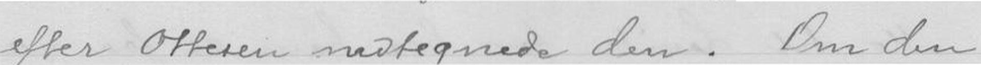efter Ottesen nedtegnede den. Om den
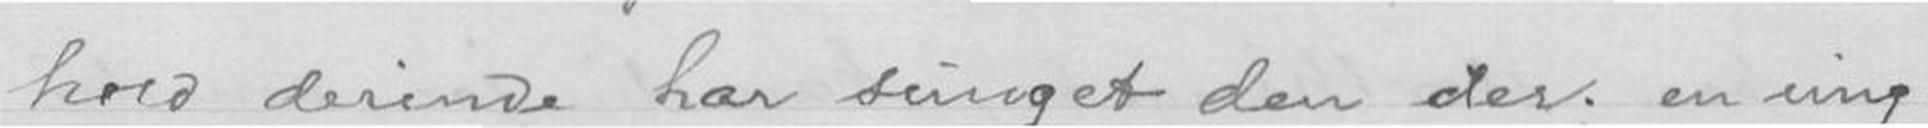hold derinde har sunget den der; en ung
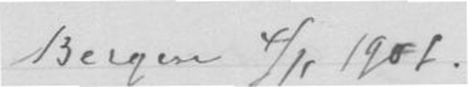Bergen 4/11 1901.
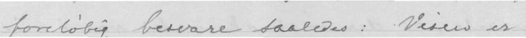foreløbig besvare saaledes: Visen er
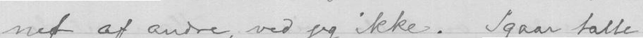net af andre, ved jeg ikke. Igaar talte
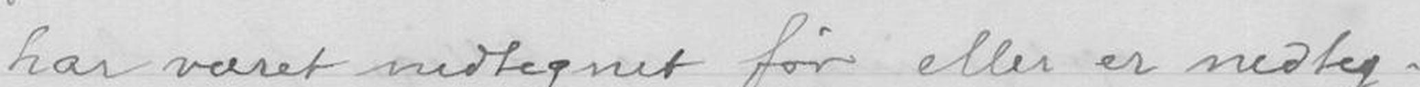har været nedtagnet før eller nedteg-
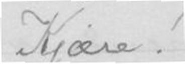Kjære!
